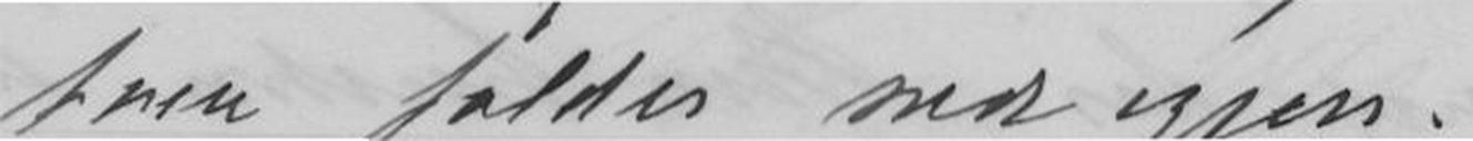toen falder ned igjen.
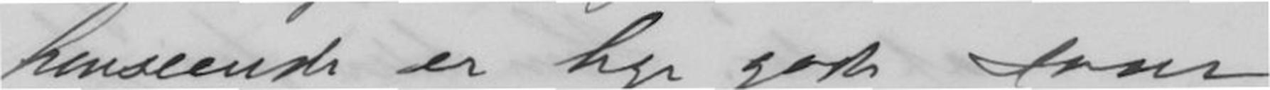henseende er lige gode som
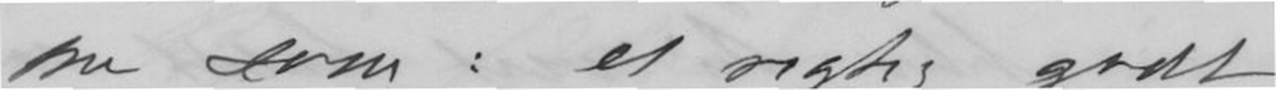me som: et rigtig godt
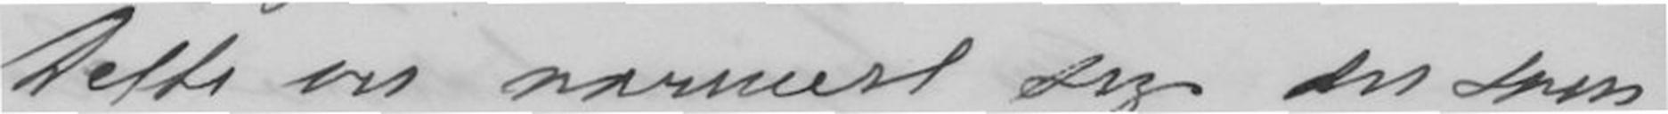Dette vil nærmest sige det sam-
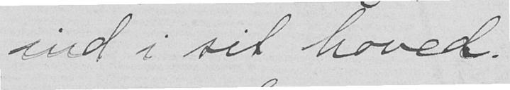ind i sit hoved.
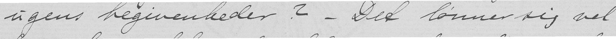ugens begivenheder? – Det lønner sig vel
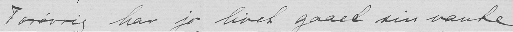Forøvrig har jo livet gaaet sin vante
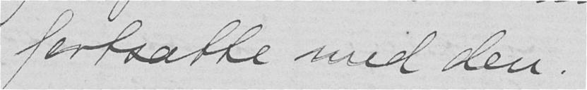fortsætte med den.
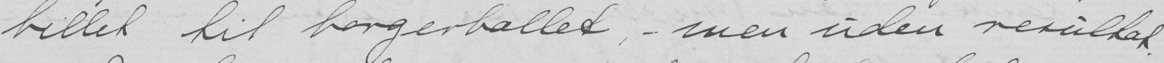billet til borgerballet, – men uden resultat.
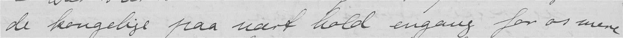de kongelige paa nært hold engang for os mere
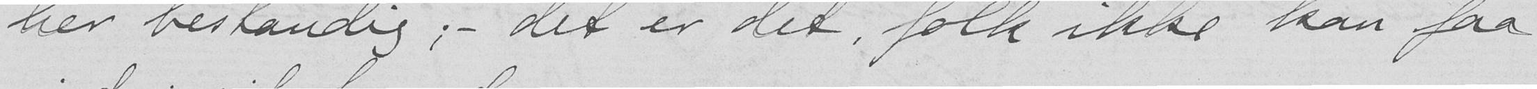her bestandig; – det er det, folk ikke kan faa
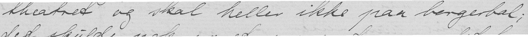theatret og skal heller ikke paa borgerbal;
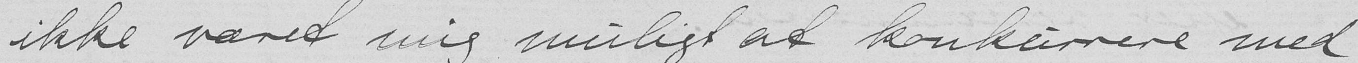ikke været mig muligt at konkurrere med
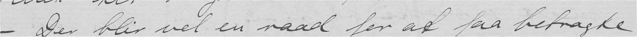– Der blir vel en raad for at faa betragte
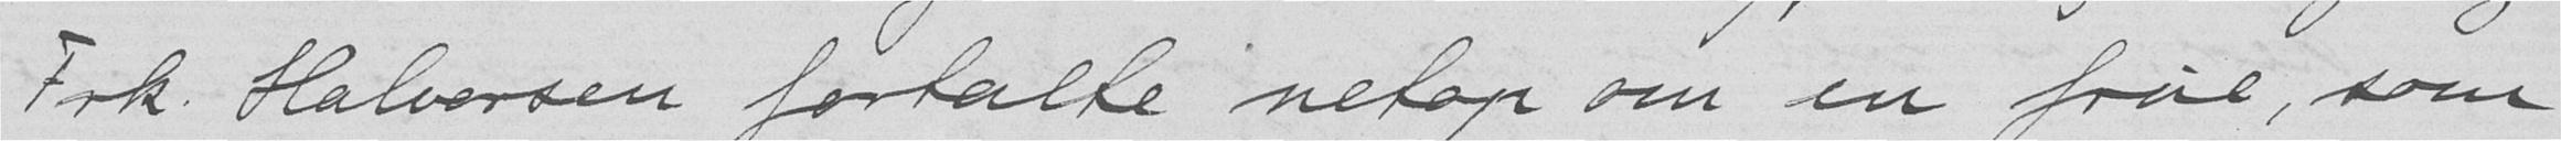Frk. Halvorsen fortalte netop om en frue, som
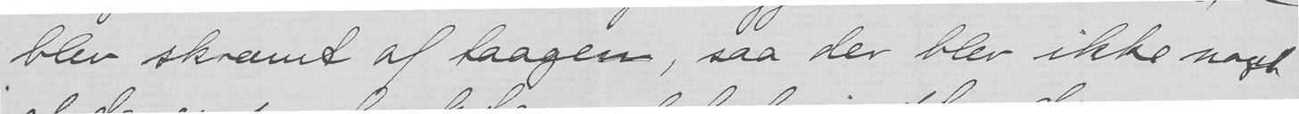blev skræmt af taagen, saa der blev ikke noget
